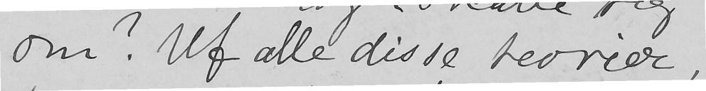om? Uf alle disse teorier,
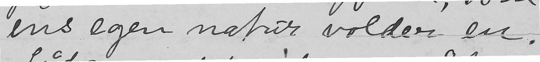ens egen natur volder en.
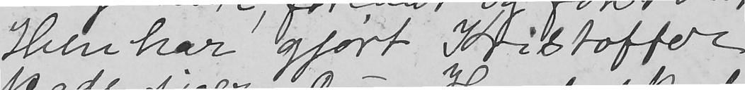Hun har gjort Kristoffer
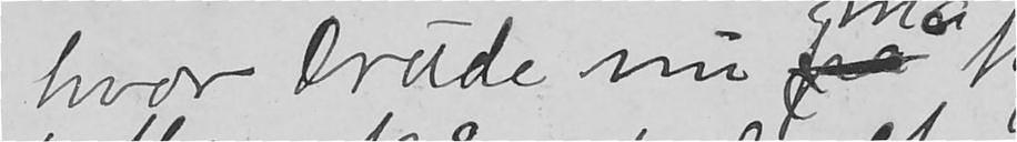hvor Drude nu
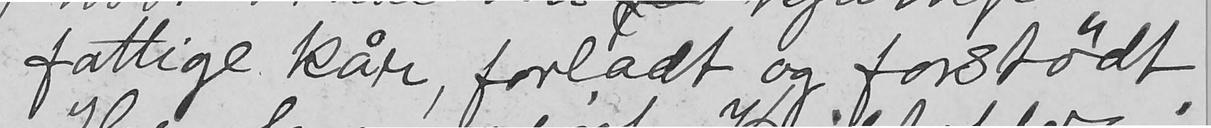fattige kår, forladt og forstødt.
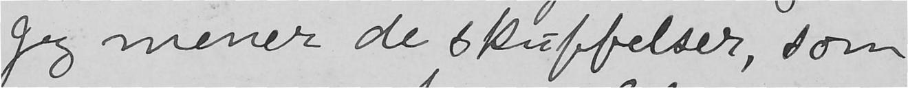Jeg mener de skuffelser, som
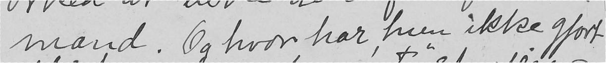mand. Og hvor har, hun ikke gjort
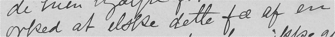orked at elske dette fæ af en
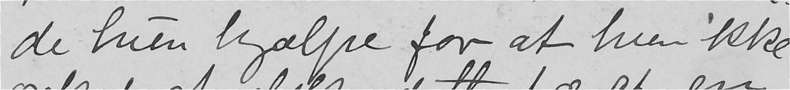de hun  hjælpe for at hun ikke
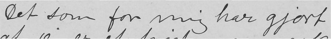Det som for mig har gjort
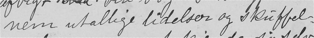nem utallige lidelser og skuffel-
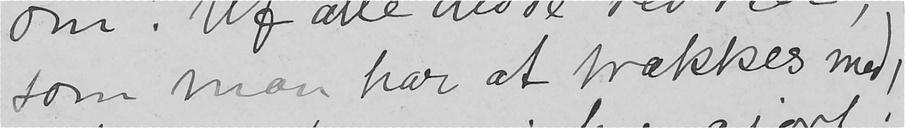som man har at trækkes med!
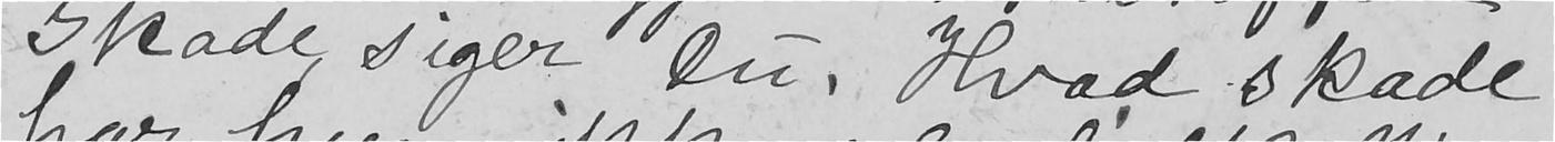skade, siger Du. Hvad skade
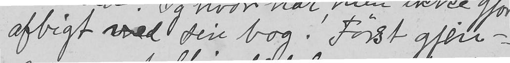afbigt ved sin bog. Først gjen-
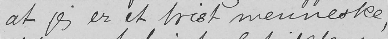at jeg er et trist menneske,
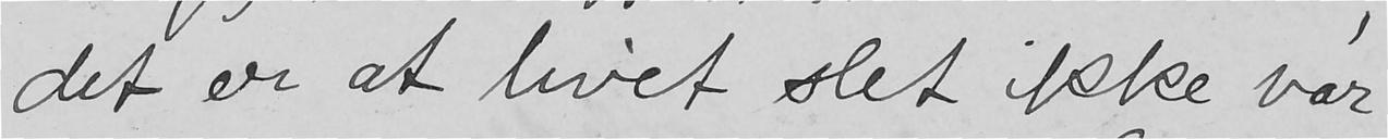det er at livet slet ikke var
